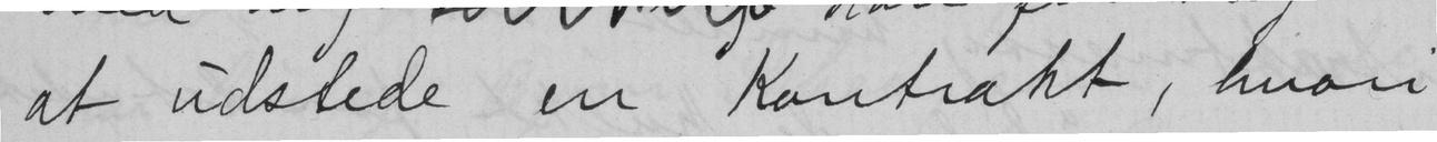at udstede en Kontrakt, hvori
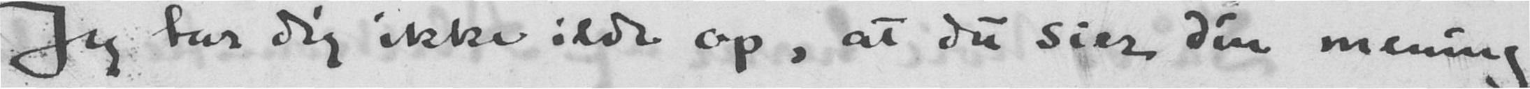Jeg tar dig ikke ilde op, at du sier din mening
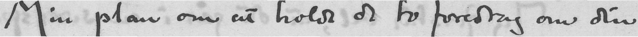Min plan om at holde de to foredrag om din
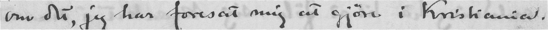om det, jeg har foresat mig at gjøre i Kristiania.
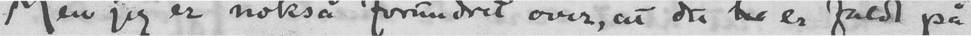Men jeg er nokså forundret over at du har er faldt på
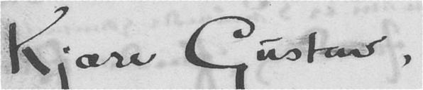Kjære Gustav,
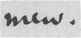men.
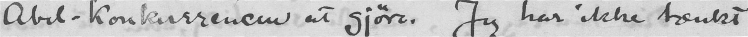Abel-konkurrencen at gjøre. Jeg har ikke tænkt
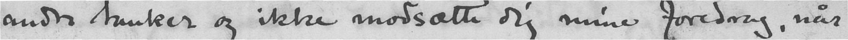andre tanker og ikke modsætte dig mine foredrag, når
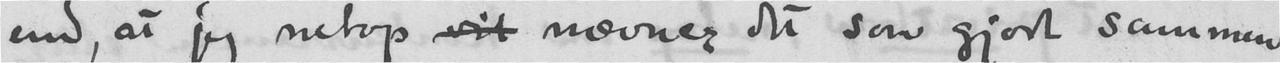end, at jeg netop vil nævner det som gjort sammen
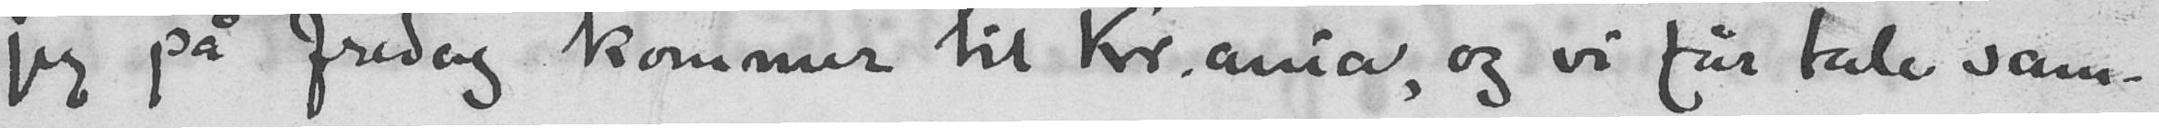jeg på fredag kommer til Kr.ania, og vi får tale sam-
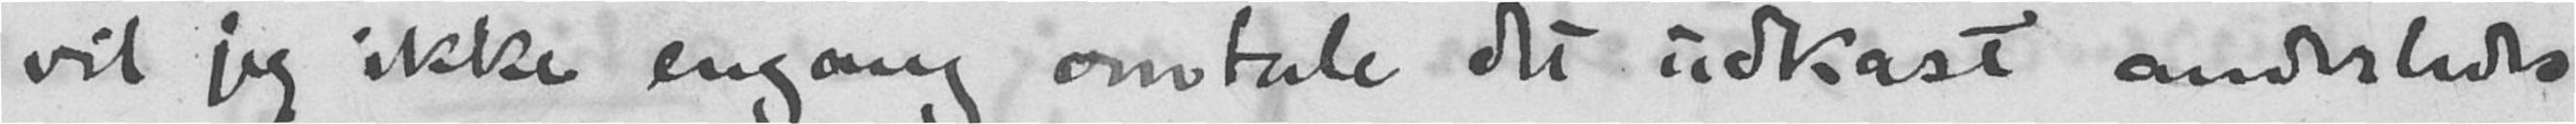vil jeg ikke engang omtale det udkast anderledes
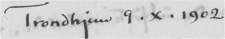Trondhjem 9.X.1902
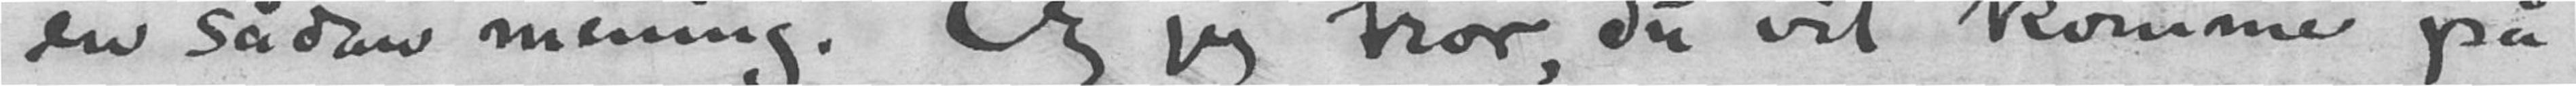en sådan mening. Og jeg tror, du vil komme på
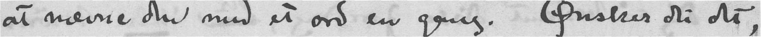at nævne den med et ord en gang. Ønsker du det,
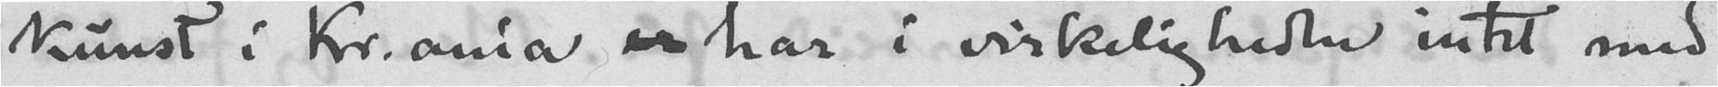kunst i Kr.ania er har i virkeligheden intet med
